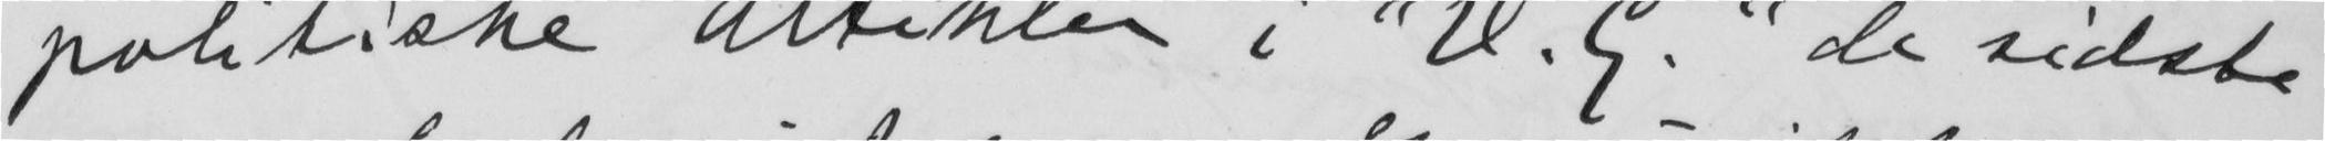politiske artikler i "V. G." de sidste
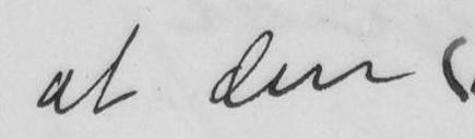at den
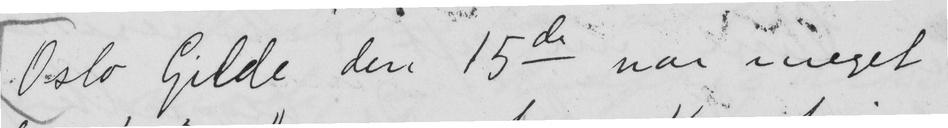Oslo Gilde den 15de var meget
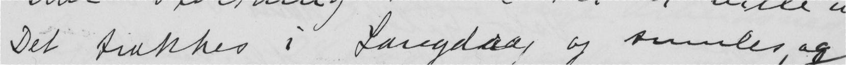Det trakkes i Langdrag og smules, og
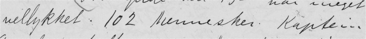vellykket. 102 Mennesker. Kaptein
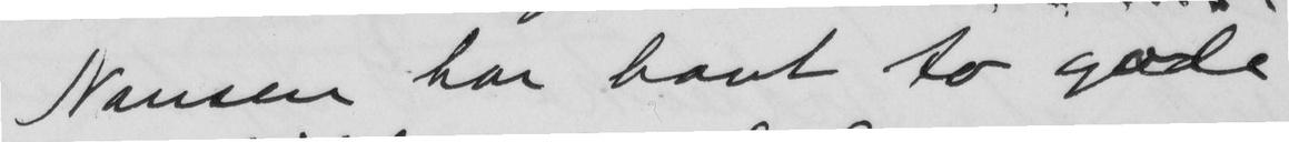Nansen har havt to gode
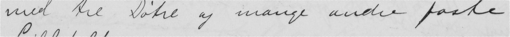med tre Døtre og mange andre faste
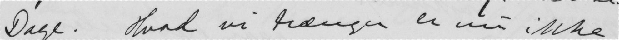Dage. Hvad vi trænger er nu ikke
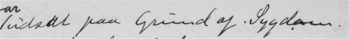udsdt paa Grund af sygdom.
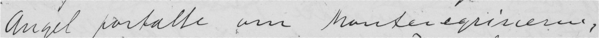Angel fortalte om Montenegrinerne,
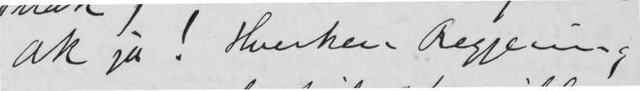Ak ja! Hverken Regjering
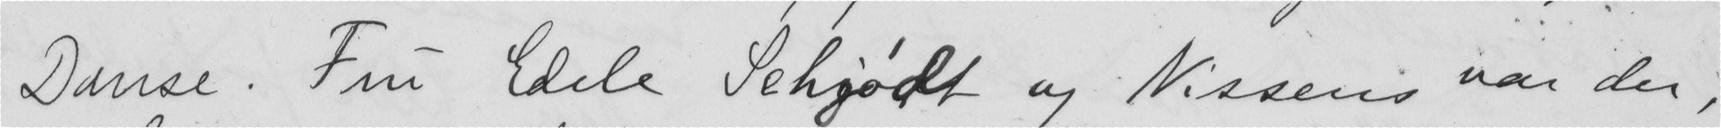Danse. Fru Edele Schjødt og Nissens var der,
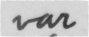var
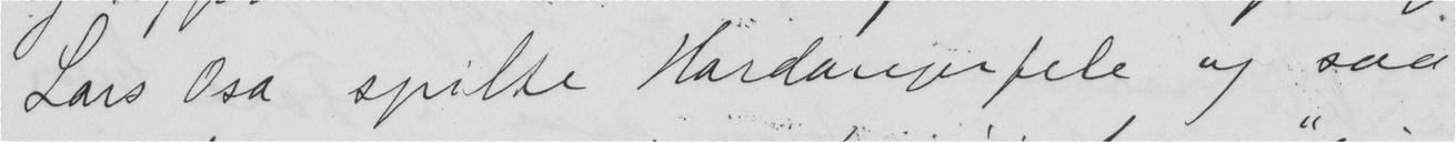Lars Osa spilte Hardangerfele og saa
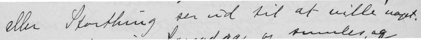eller Storthing ser ud til at ville noget.
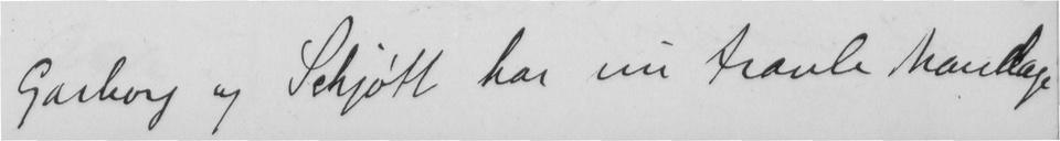Garborg og Schjøtt har nu travle Mandager
